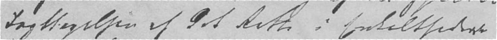Iagttagelsen af det Rette i Enkeltheder
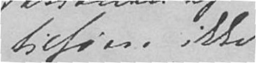tilhører ikke
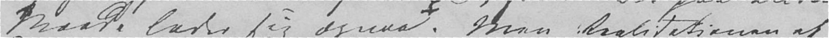Maade lader sig opnaa. Men Realisationen af
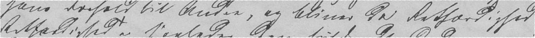hans Forhold til Andre, og bliver da Retfærdighed.
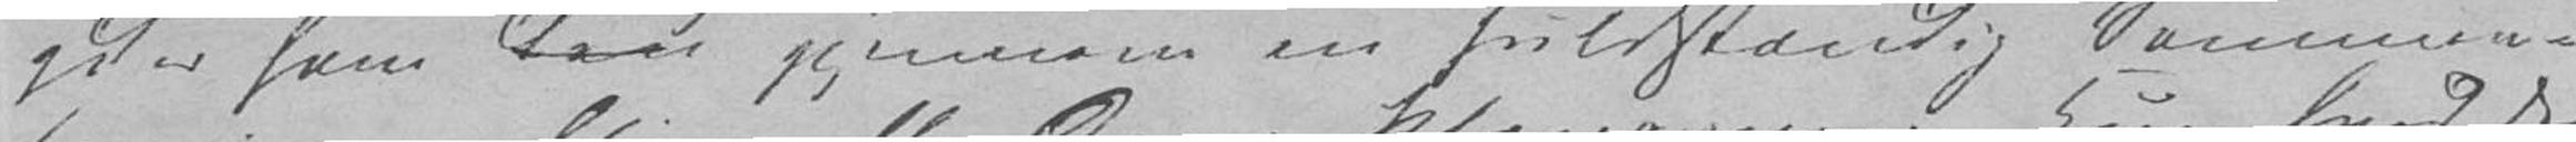yder ham den gjennem en fuldstændig Sammen-
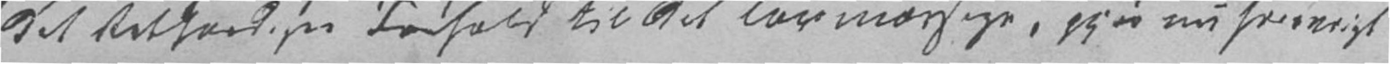det Retfærdiges Forhold til det Lovmæssiges, gjør nu forøvrigt
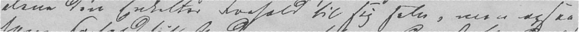alene den Enkeltes Forhold til sig selv, men ogsaa
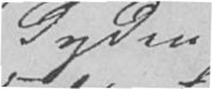Dyden
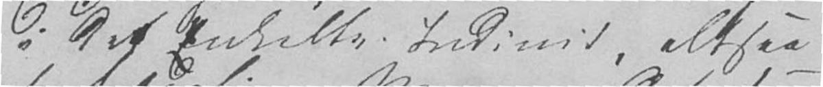i det enkelte Individ, altsaa
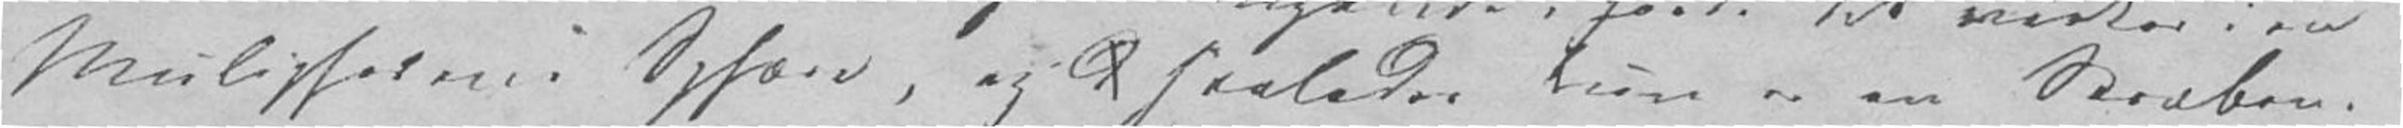Mulighedens Sphære, og d saaledes kun er en Stræben.
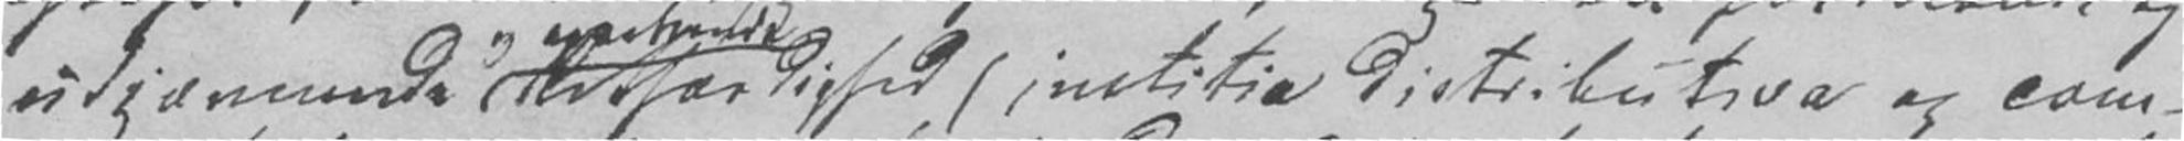udjævnende Redfærdighed (iustitia distributiva og com-
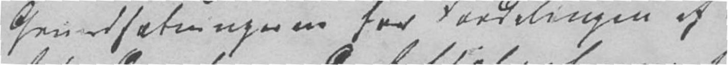Grundsætningerne for Fordelingen af
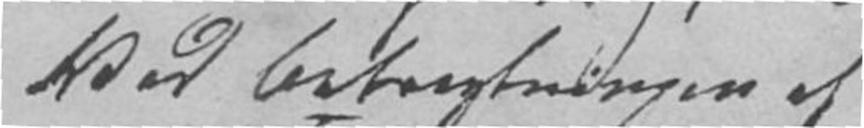Ved Betragtningen af
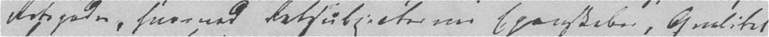Retsgoder, hvorved Retsubjecternes Egenskaber, Qvalitet
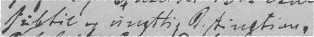subtil og unyttig Distinction.
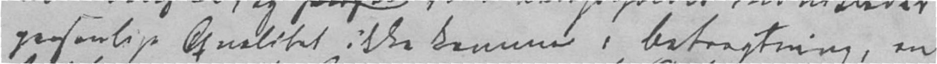personlige Ovalitet ikke kommer i Betragtning, en
